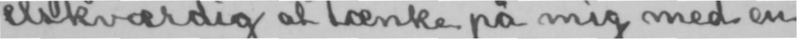elskværdig at tænke på mig med en
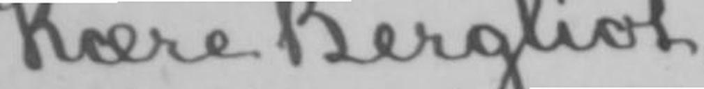Kære Bergliot,
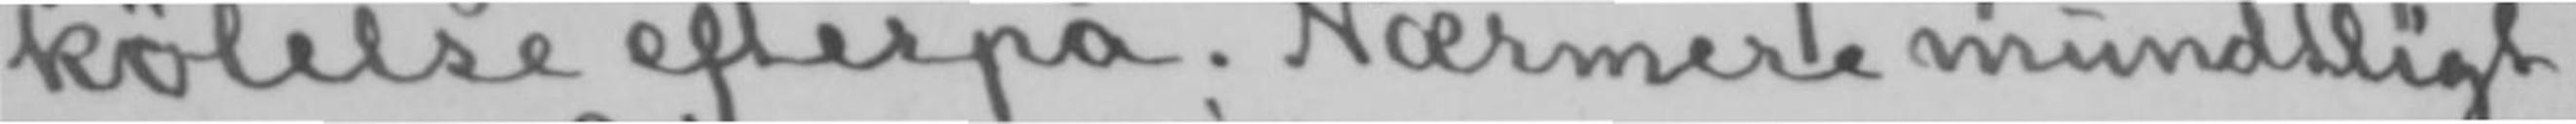kølelse efterpå. Nærmere mundtligt.
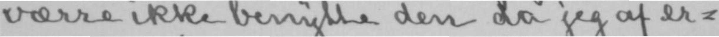værre ikke benytte den da jeg af er-
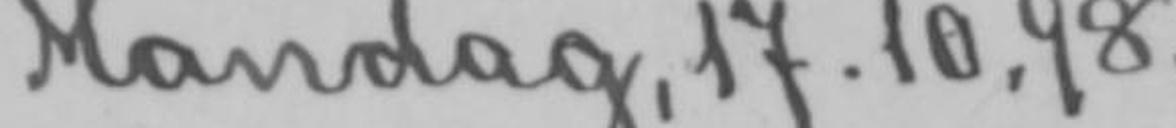Mandag, 17.10.98.
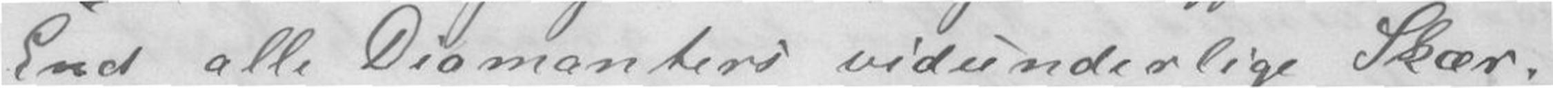End alle Diamanters vidunderlige Skær.
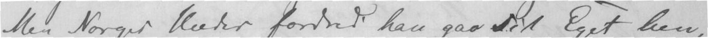Men Norges Hæder fordred han gav sit Eget hen,
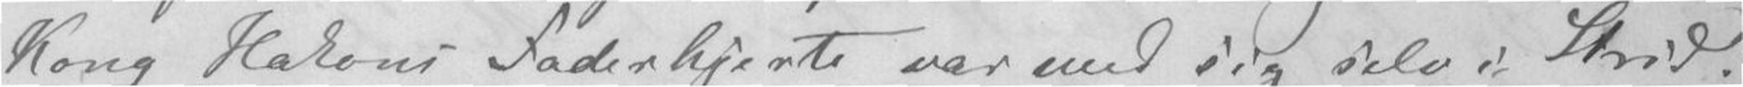Kong Håkons Faderhjerte var med sig selv i Strid.
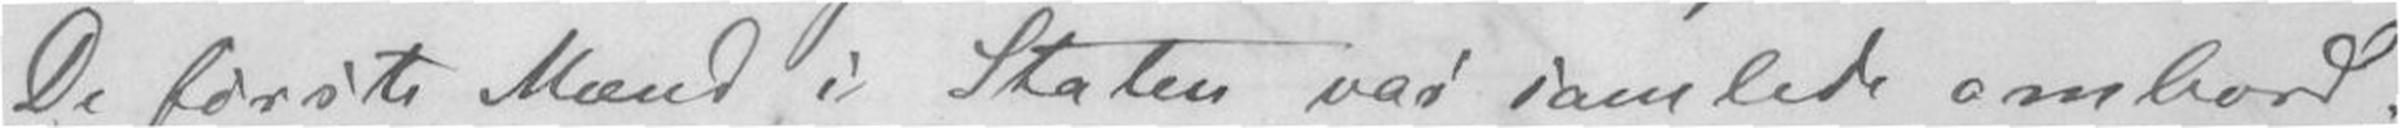De første Mænd i Staten var samlede ombord;
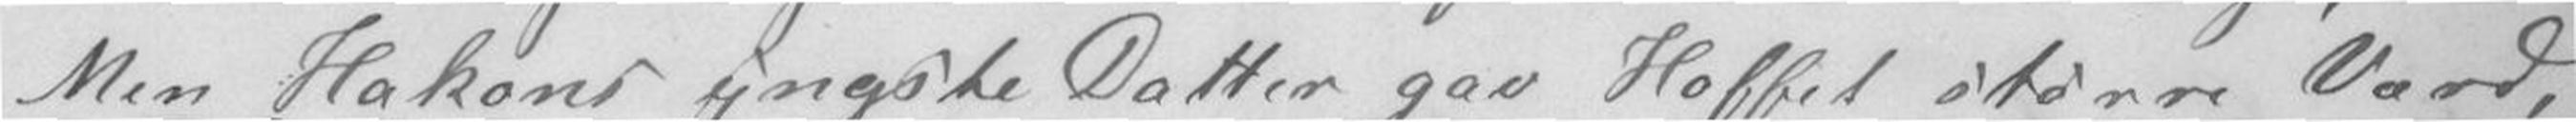Men Håkons yngste Datter gav Hoffet større Værd,
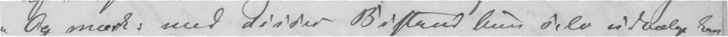"Og mark med disses Bistand hun selv vil vælge ham
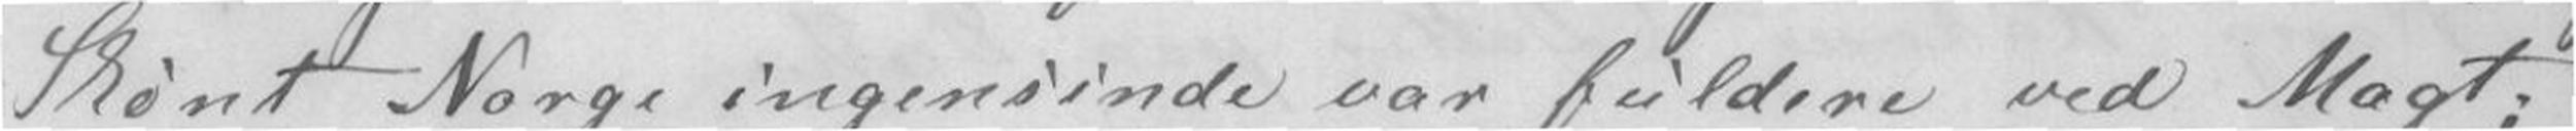Skønt Norge ingensinde var fuldere ved Magt;
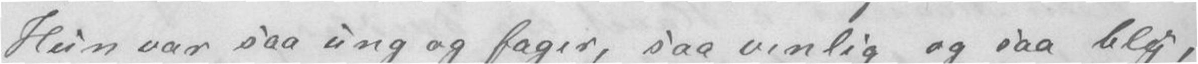Hun var saa ung og fager, saa venlig og saa blyg,
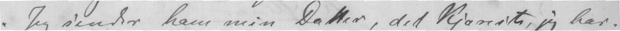"Jeg sender ham min Datter, det Kjæreste, jeg har.
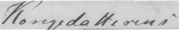Kongedatterens
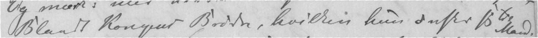"Blandt Kongens Brødre, hvilken hun ønsker B til Mand.
